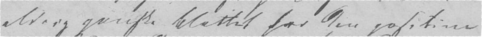aldrig ganske blottet for den positive
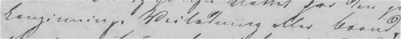Lovgivningens Veiledning eller Baand,
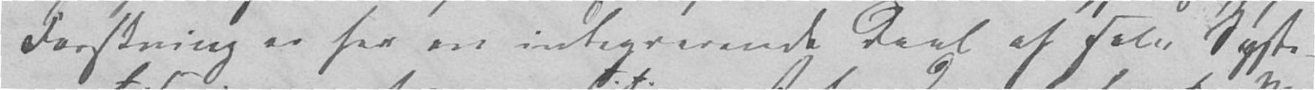Forskning er her en integrerende Deel af selve Syst-
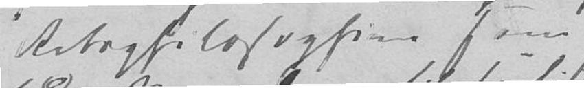Retsphilosophien som
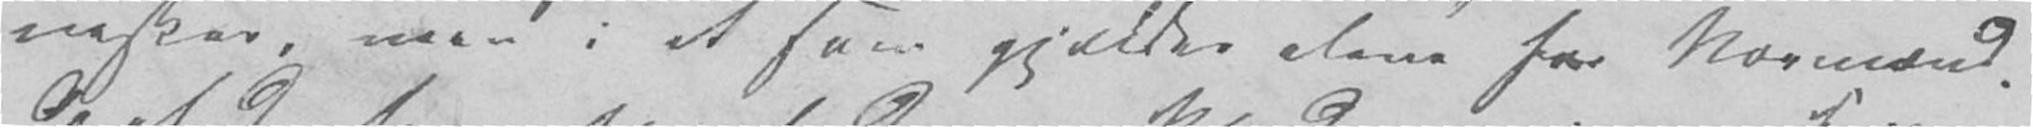nesker, men i et som gjælder alene for Normænd.
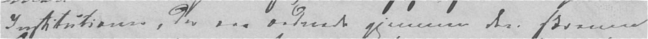Institutioner, der ere ordnede gjennem den skrevne
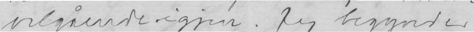velgående igjen. Jeg begynder
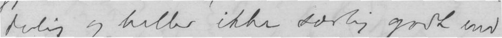delig og heller ikke særlig godt end
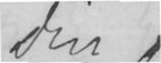Din
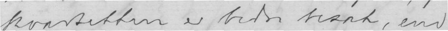kvartetten er bedre besat, end
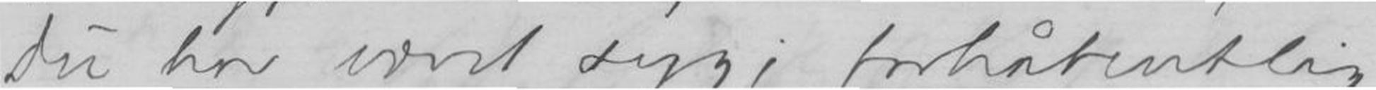du har vært syg; forhåbentlig
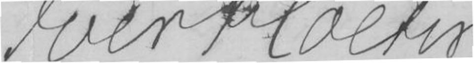Iver Holter
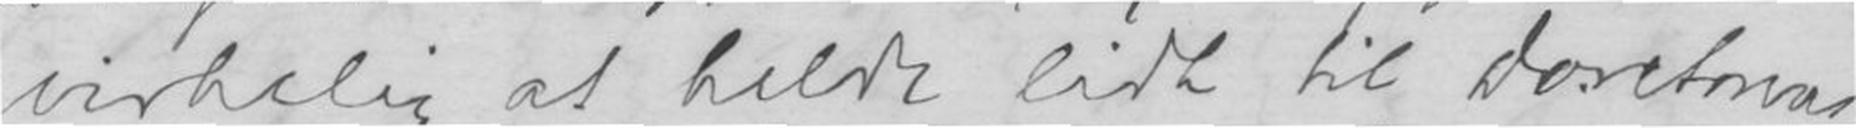virkelig at heldt lidt til Dorctorens
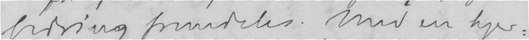bedring fremdeles. Med en hjer-
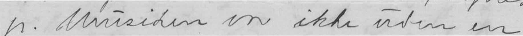jeg. Musiken var ikke uden en
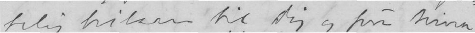telig hilsen til dig og fru Nina
